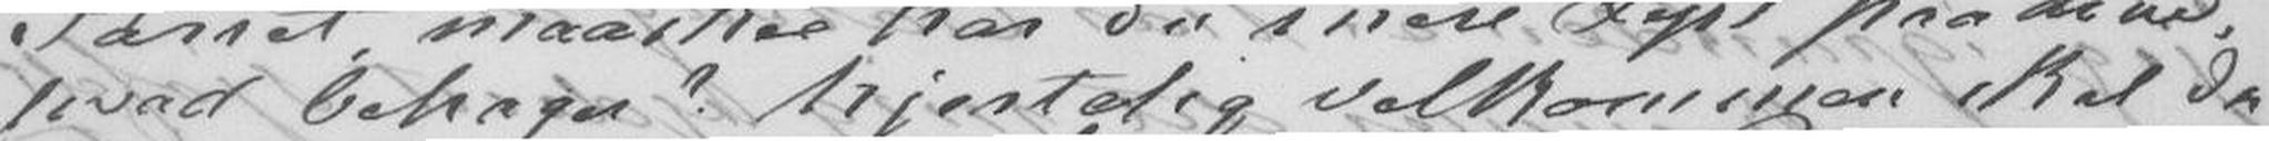hvad behager? hjertelig velkommen skal Du
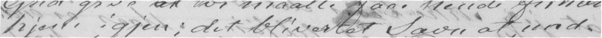hjem igjen; det bliver let Savn at und-
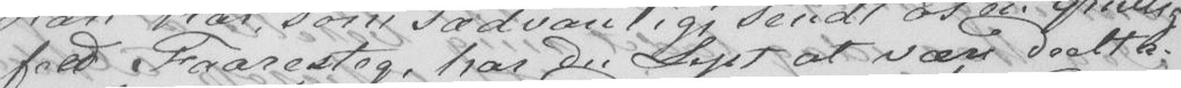feed Faaresteg, har Du Lyst at være Deelta-
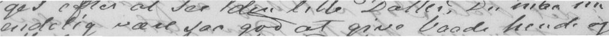endelig være saa god at give baade hende og
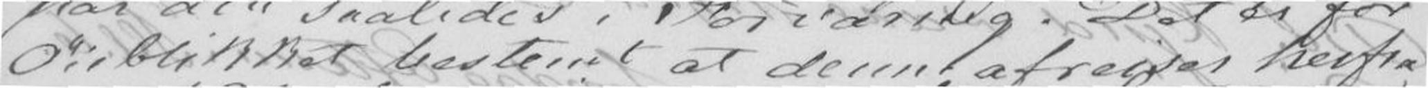Øieblikket bestemt at denne afreiser herfra
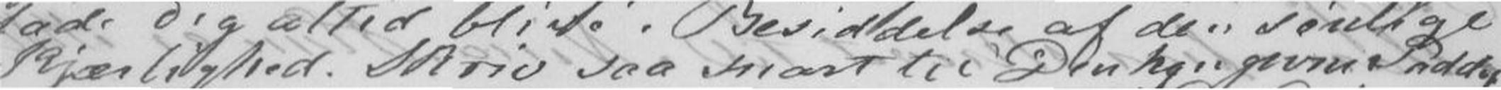Kjærlighed. Skriv saa snart til Din hengivne Paddy
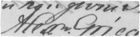Alexn Grieg.
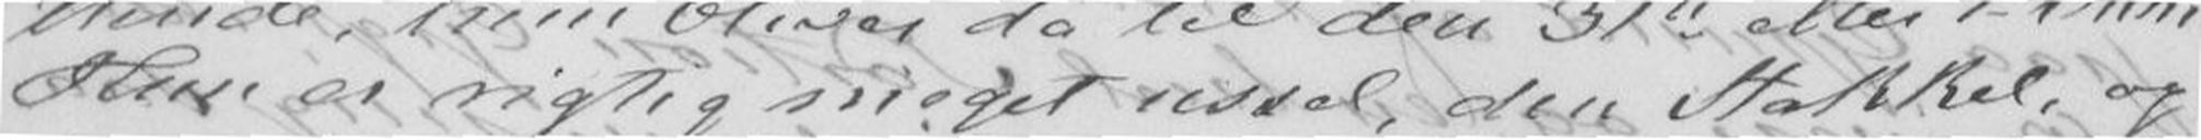Hun er rigtig meget ussel, den Stakkel og
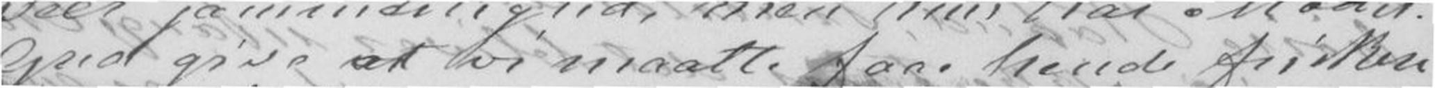Gud give at vi maatte faae hende friskere
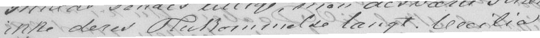ikke deres Hukommelse langt. Cecilia
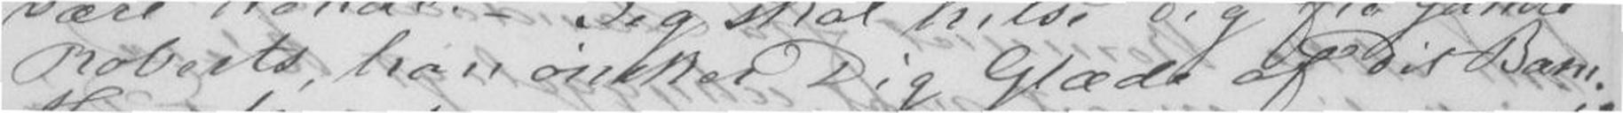Roberts, han ønsket Dig Glæde af Dit Barn.
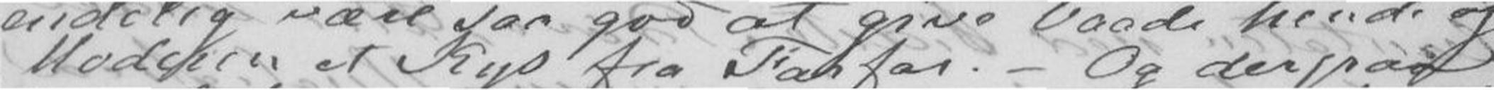Moderen at Kys fra Farfar. - Og der paa
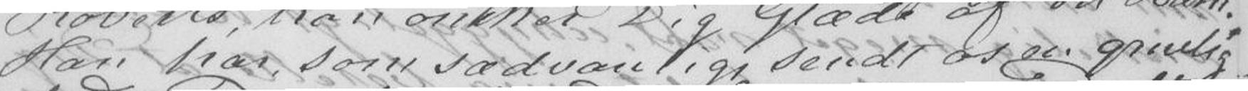Han har som sædvanligs sendt os en gruelig
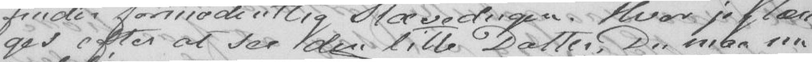ges efter at see den lille Datter. Du maa nu
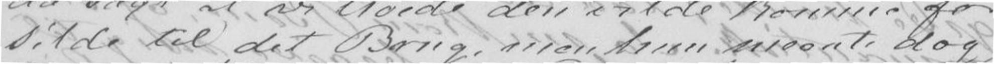silde til det Brug, men hun meente dog
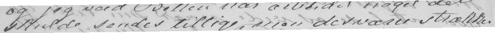skulde sendes tillige, men desværre strække
